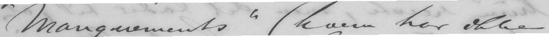"Manquements" (hvem har ikke
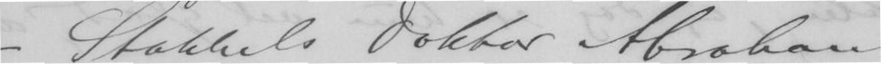- Stakkels Doktor Abraham
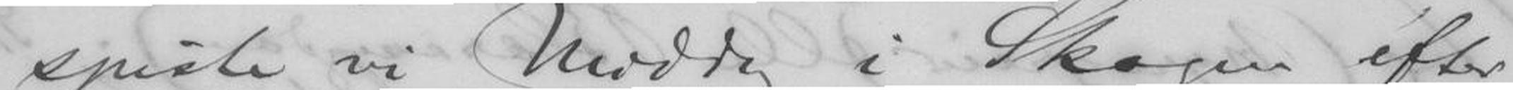spiste vi Middag i Skogen efter
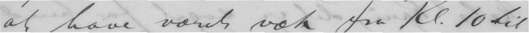at have været væk fra Kl.10 til
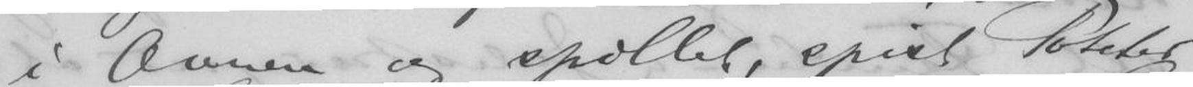i Ovnen og spillet, spist Poteter
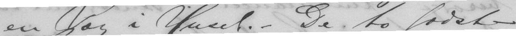en Væn i Huset. - De to sidste
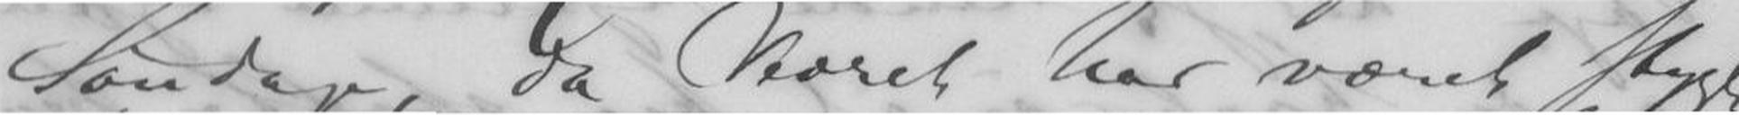Søndage, da Veiret har været stygt,
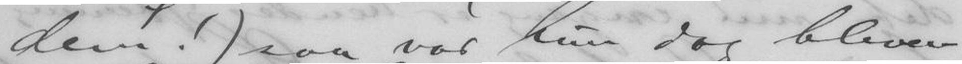dem!) saa var hun dog bleven
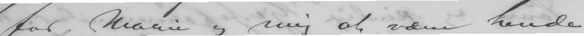for Marie og mig at være hende
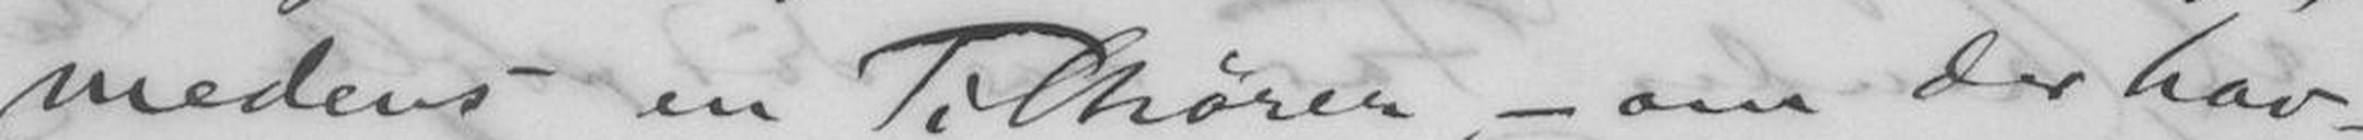medens en Tilhører, - om der hav-
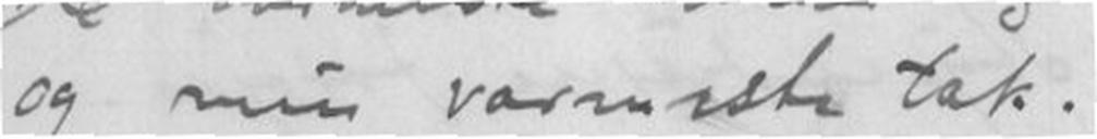og min varmeste tak.
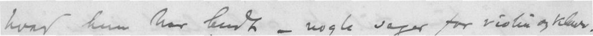hvad hun har budt - nogle sager for violin og klaver.
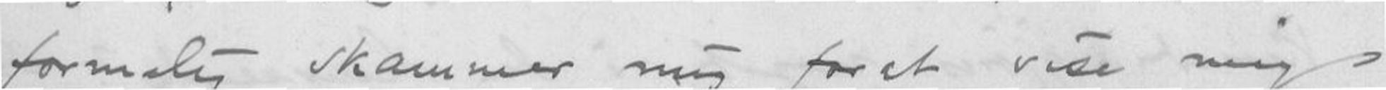formelig skammer mig for at vise mig
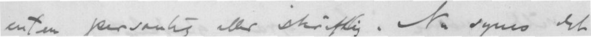enten personlig eller skriftlig. Nu synes det
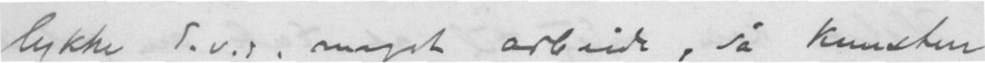lykke d.v.s. meget arbeide, så kunsten
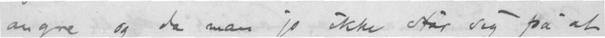angre og da man jo ikke står sig på at
